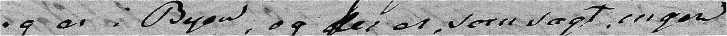jeg er i Byen, og der er som sagt ingen
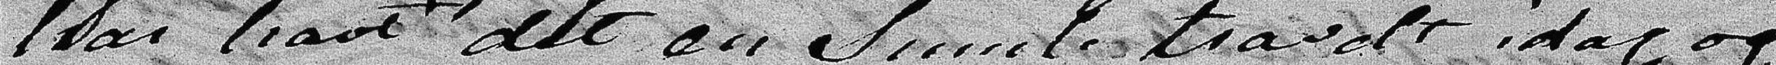har havt det en Smule travelt idag og
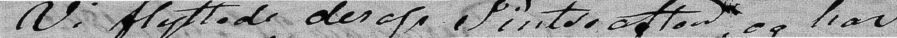Vi flyttede derop Pintseaften og har
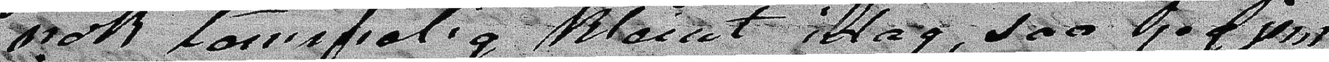nok temmelig kleint idag, saa jeg just
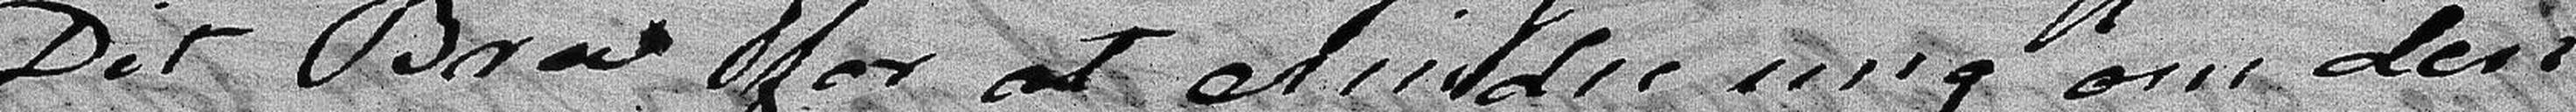Dit Brev for at erindre mig om deri
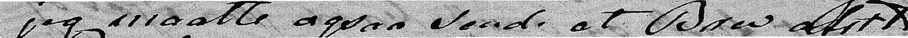jeg maatte ogsaa sende et Brev afsted
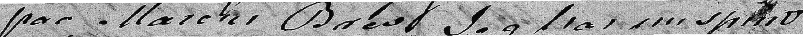paa Marens Brev. Jeg har nu spiist
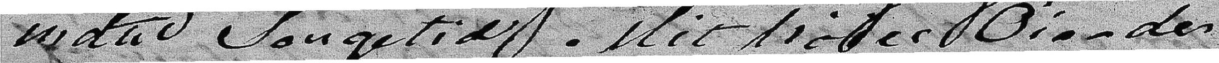indtil Sengetid. Mit høire Øie der
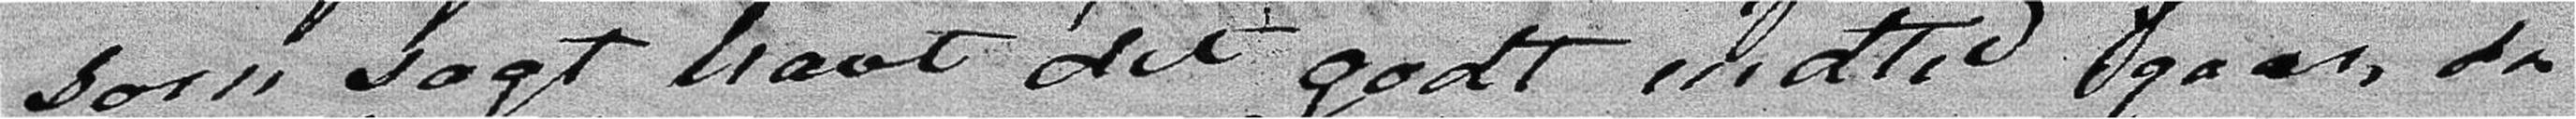som sagt havt det godt indtil igaar, da
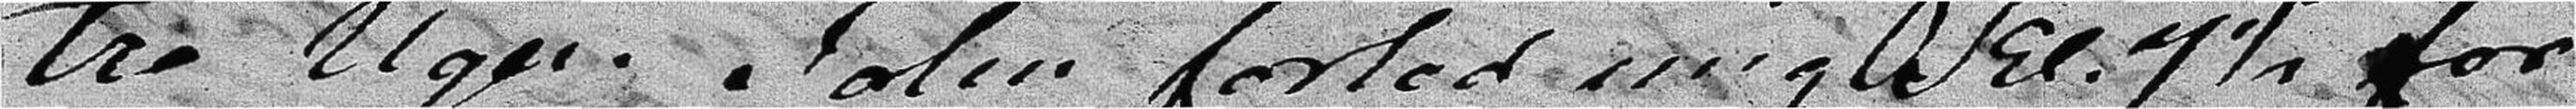tre Uger. John forlod mig Kl.7½. for
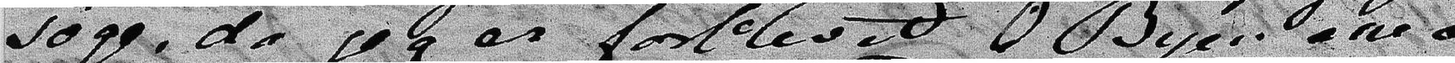søge, da jeg er forblevet i Byen ene og
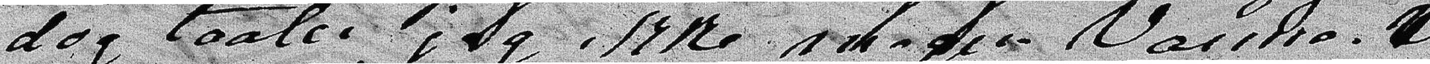dog taaler jeg ikke megen Varme. Vi
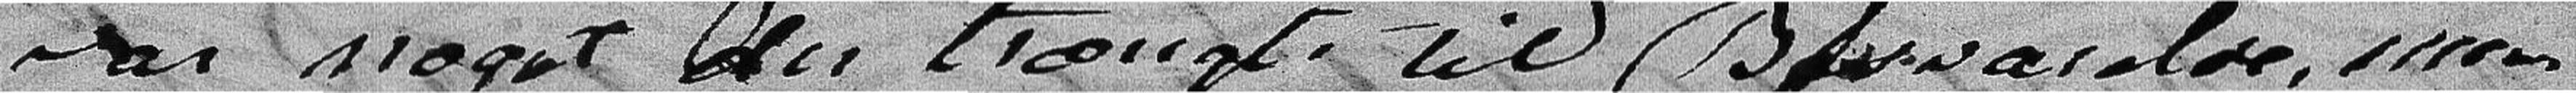var noget der trængte til Besvarelse, men
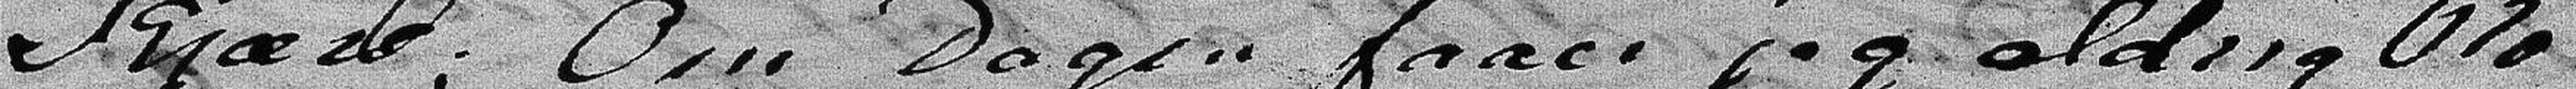Kjære. Om Dagen faaer jeg aldrig Ro
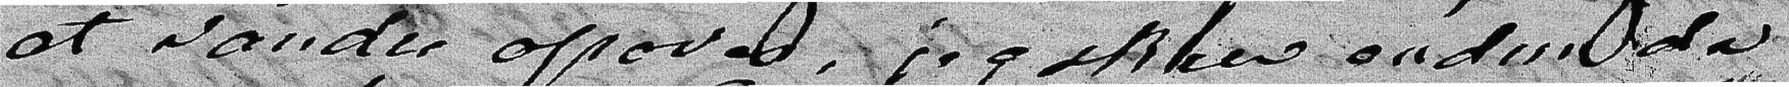at vandre opover, jeg skrev endnu da
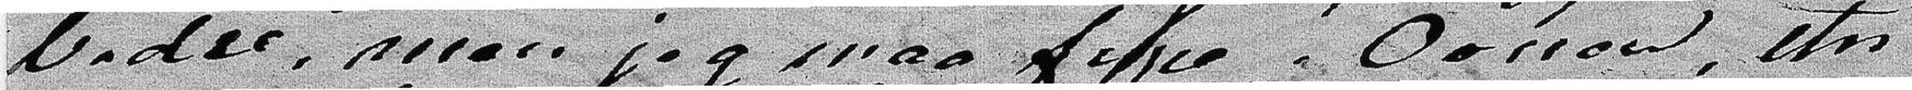bedre, men jeg maa fyre i Ovnen, thi
